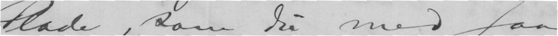Plade, som du med saa
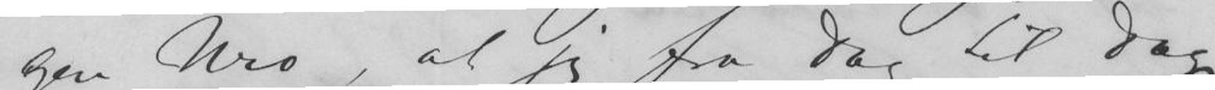gen Uro, at jeg fra Dag til Dag
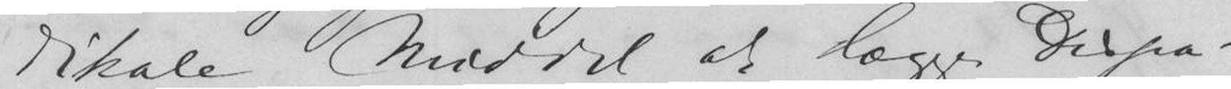dikale Middel at lægge Dispa-
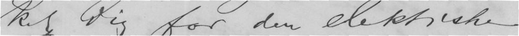ket dig for den elektriske
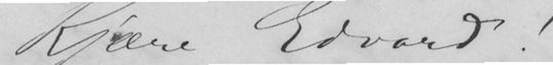Kjære Edvard!
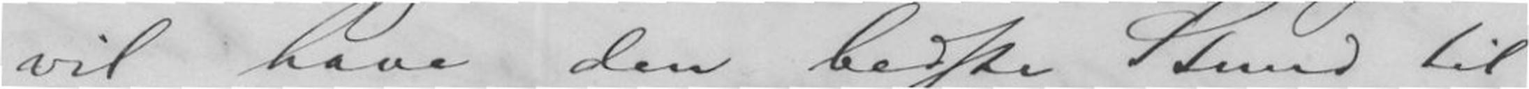vil have den bedste Stund til
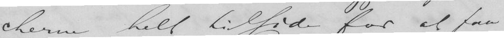cherne helt tilside for at faa
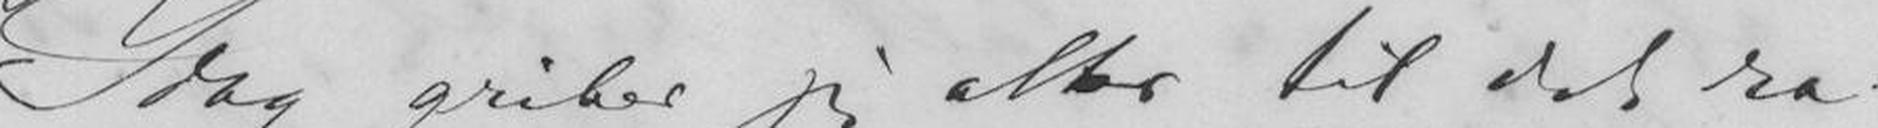Idag griber jeg atter til det ra-
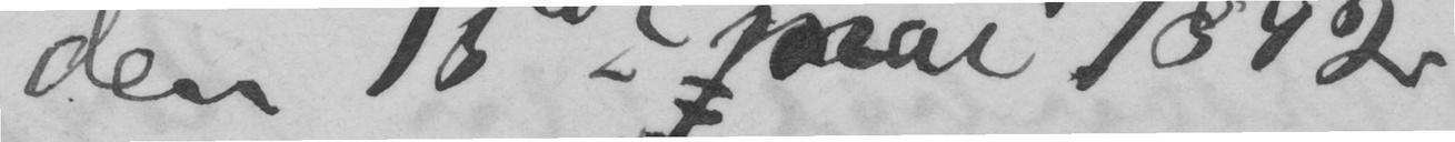den 15de mai 1892
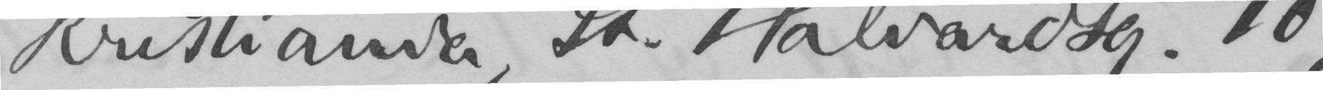Kristiania, St. Halvardsg. 10
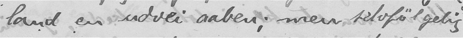land en udvei aaben; men selvfølgelig
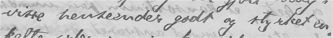visse henseender godt og styrket en-
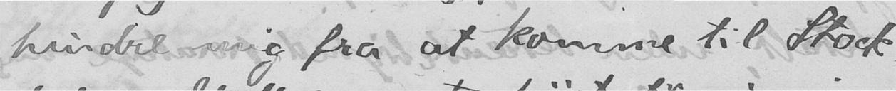hindre mig fra at komme til Stock-
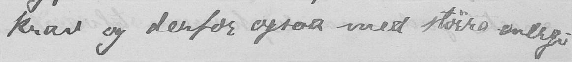krav og derfor ogsaa med større energi
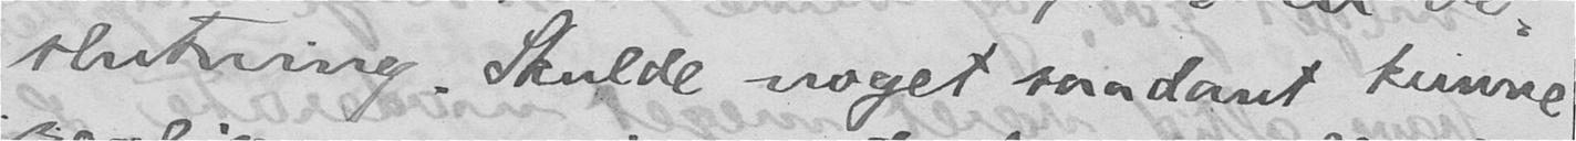slutning. Skulde noget saadant kunne
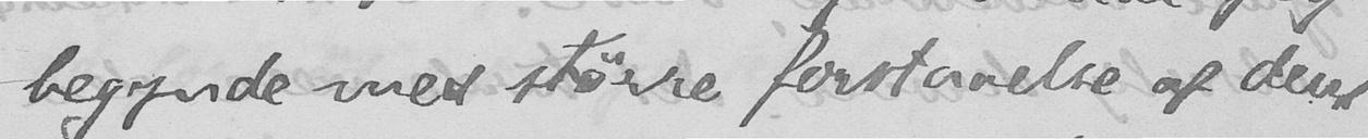begynde med større forstaaelse af dens
